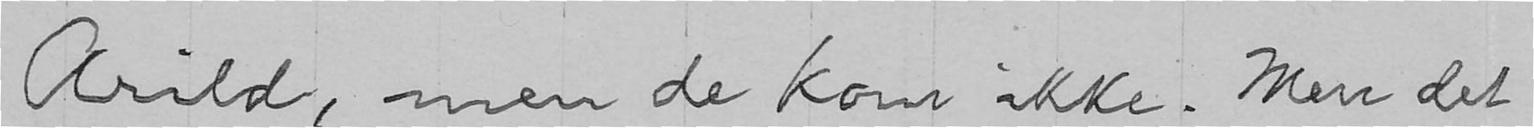Arild, men de kom ikke. Men det
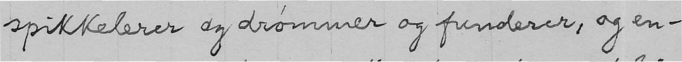spikkelerer og drømmer og funderer, og en-
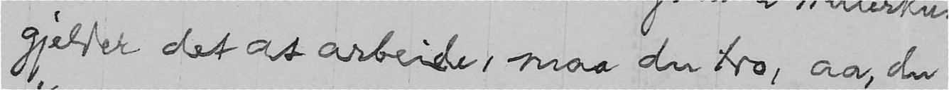gjelder det at arbeide, maa du tro, aa, du
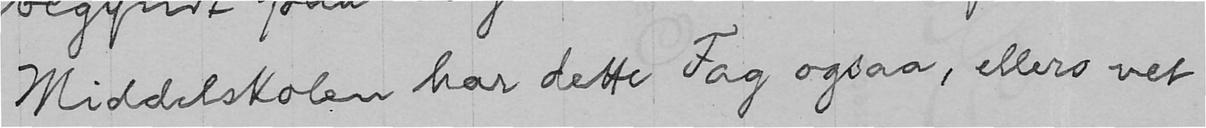Middelskolen har dette Fag ogsaa, ellers vet
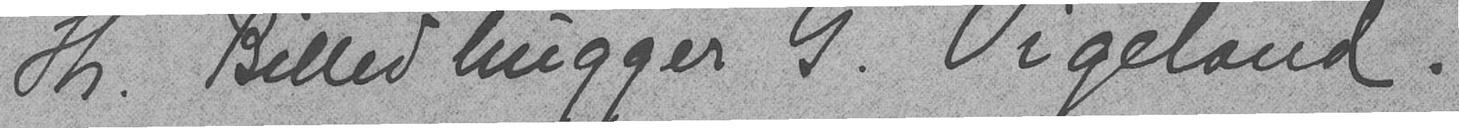Hr. Billedhugger G. Vigeland
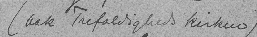(bak Trefoldighedskirken)
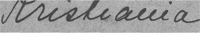Kristiania
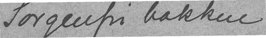Sorgenfribakken
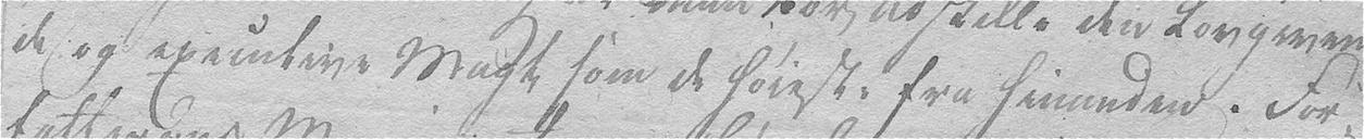de og executive Magt som de høieste fra hinanden. For-
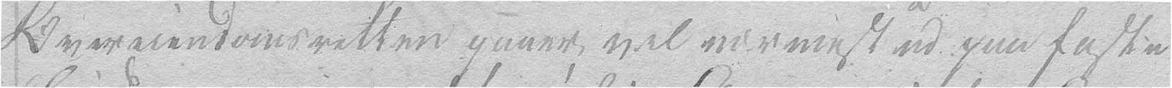Overeiendomsretten gaaer vel nærmest ud paa faste
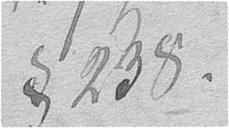§ 238.
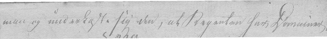man og underkaste sig den, at Regenten heri dømmer.
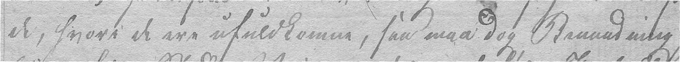de, hvori de ere ufuldkomne, saa maa dog Benaadning
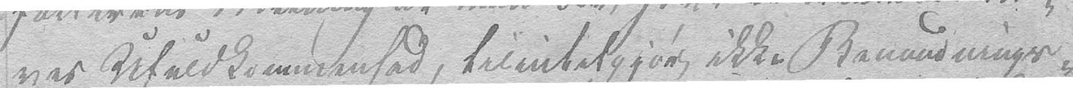ves Ufuldkommenhed, tilintetgjør ikke Benaadnings-
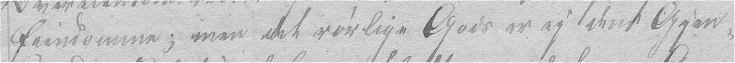Eiendomme; men det rørlige Gods er ey dens Gjen-
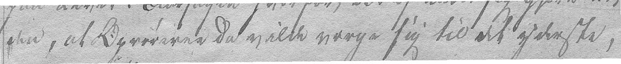den, at Oprøreren da vilde værge sig til det yderste,
